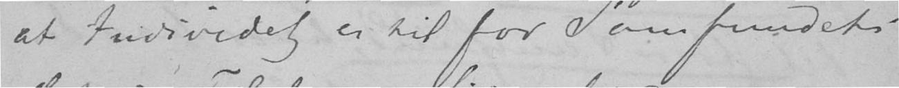at Individet er til for Samfundet
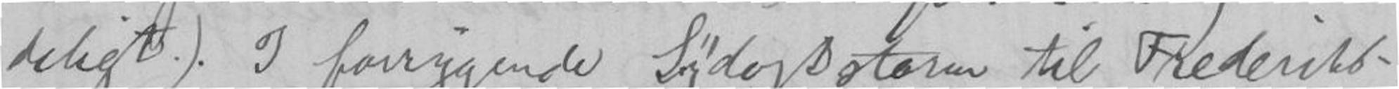deligt.) I forrygende Sydoststorm til Frederiks-
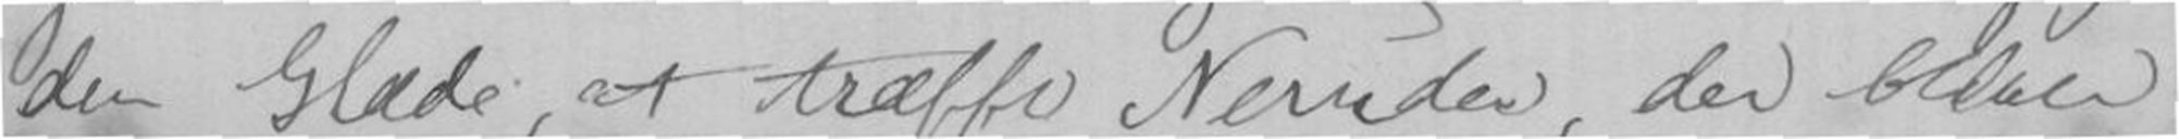den Glæde, at træffe Neruda, der bliver
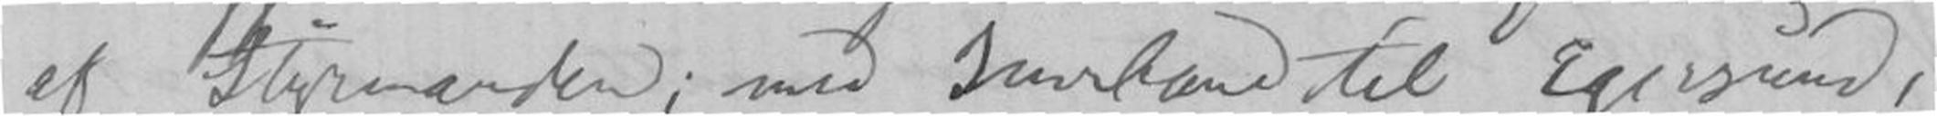af Styrmanden; med Jernbane til Egersund,
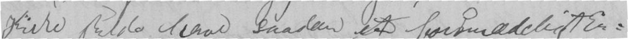Kirke skulde have saadan et forsmædeligt En-
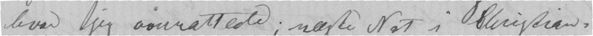hvor jeg overnattede; næste Nat i Christian-
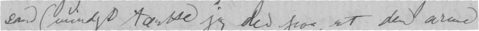sand (mindst tænkte jeg der paa, at den arme
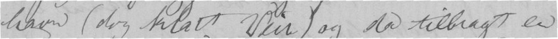havn (dog klart Veir) og da tilbragt en
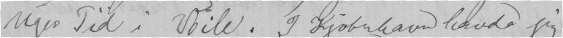Uges Tid i Veile. I Kjøbenhavn havde jeg
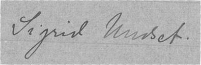Sigrid Undset.
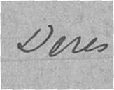Deres
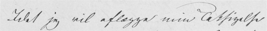Idet jeg vil aflægge min Taksigelse
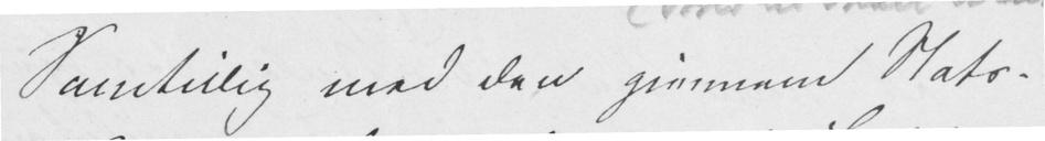Samtidig med den gjennem Stats-
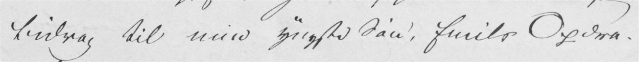Bidrag til min yngste Søn, Emils Opdra-
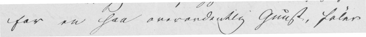for en saa overordentlig Gunst, føler
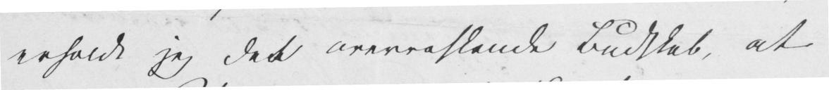erholdt jeg det overraskende Budskab, at
